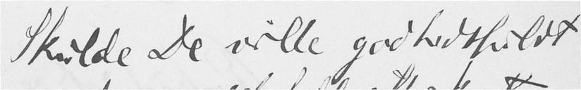Skulde De ville godhedsfuldt
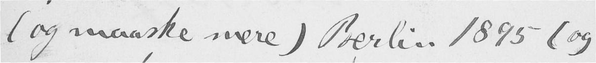(og maaske mere) Berlin 1895 (og
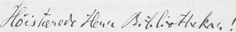Høistærede Herr Bibliothekar!
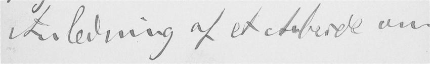Anledning af et Arbeide om
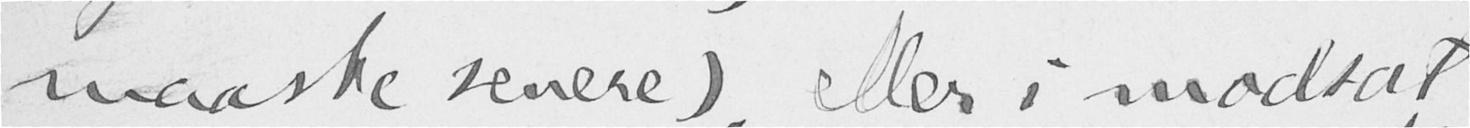maaske senere), eller i modsat
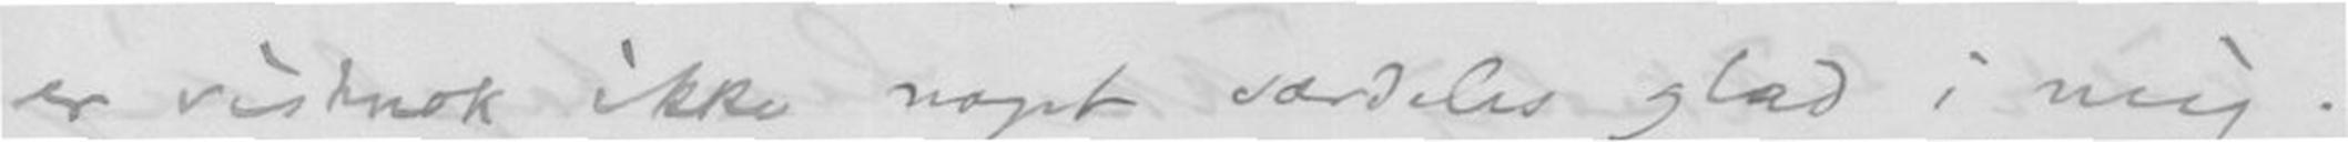er vidstnok ikke noget særdeles glad i mig.
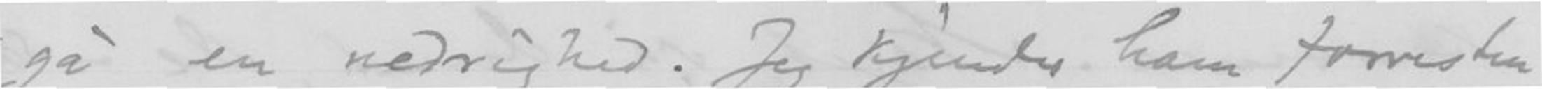gå en nedrighed. Jeg kjender ham forresten
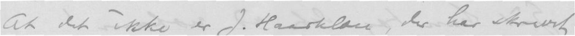At det ikke er J. Haarklou, der har skrevet
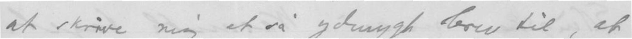at skrive mig et så ydmygt brev til, at
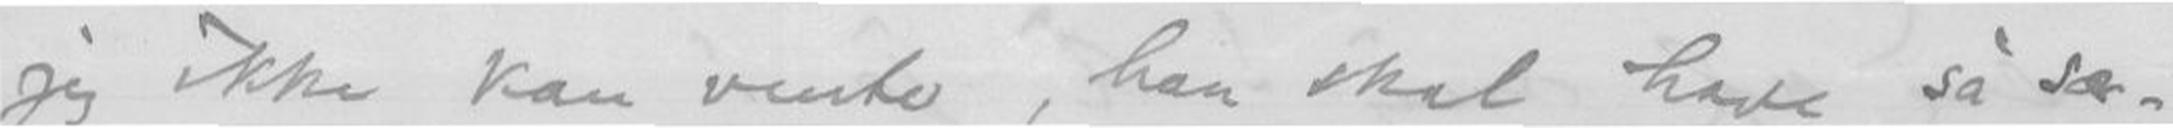jeg ikke kan vente, han skal have så sær-
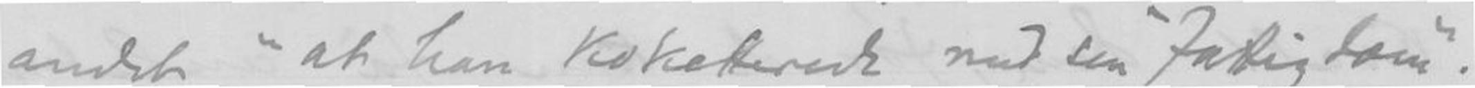andet "at han koketterede med sin fattigdom".
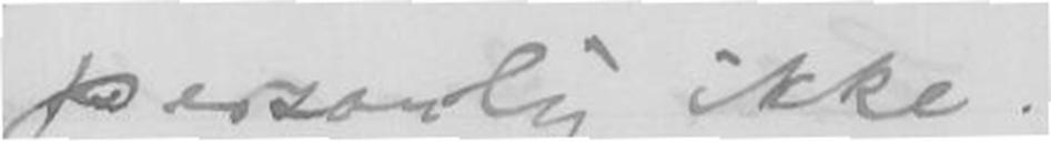personlig ikke.
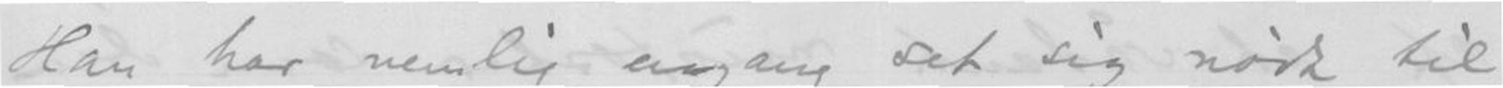Han har nemlig engang set sig nødt til
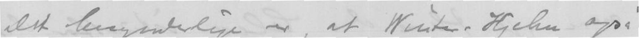Det besynderlige er, at Winter-Hjelm også
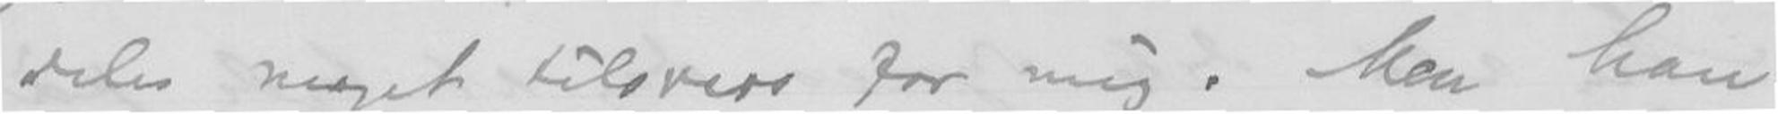deles meget tilovers for mig. Men han
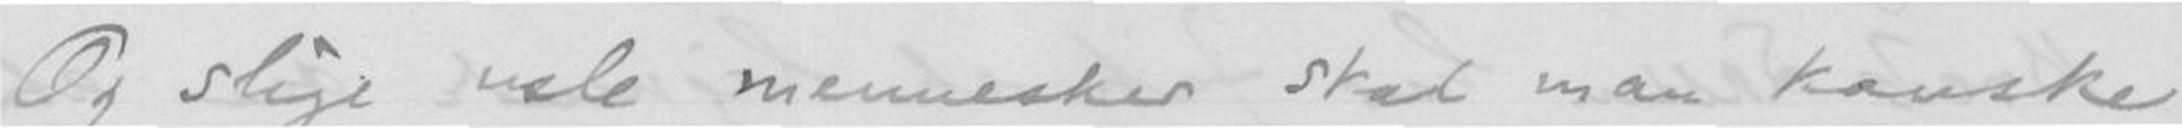Og slige usle mennesker skal man kanske
